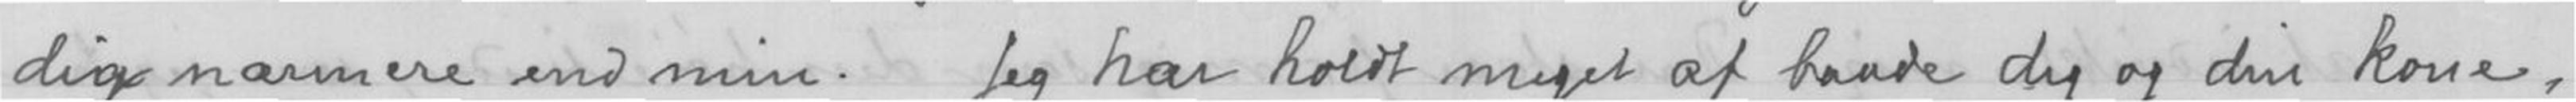dig nærmere end mine. Jeg har holdt meget af baade dig og din kone,
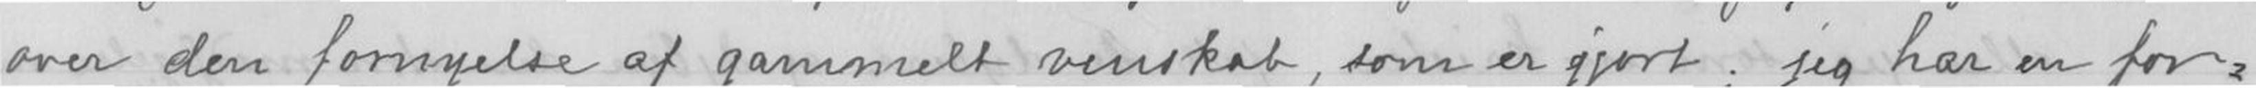over den fornyelse af gammelt venskab, som er gjort; jeg har en for-
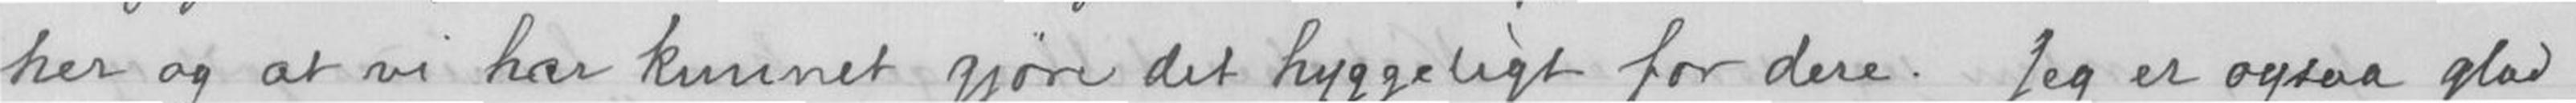her og at vi har kunnet gjøre det hyggeligt for dere. Jeg er ogsaa glad
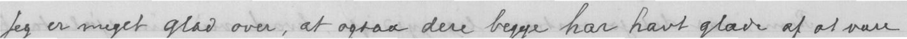Jeg er meget glad over, at ogsaa dere begge har havt glæde af at være
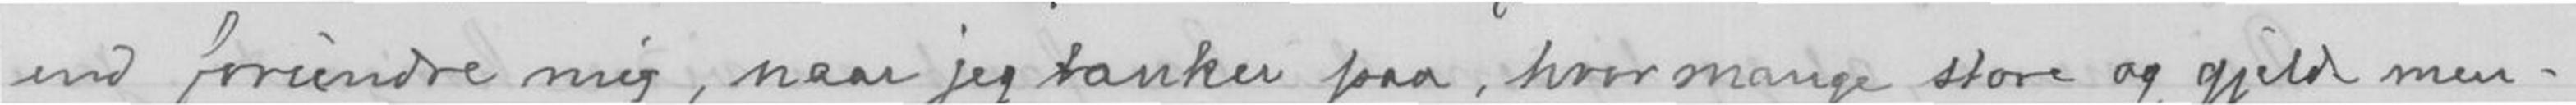end forundre mig, naar jeg tænker paa, hvor mange store og gjilde men-
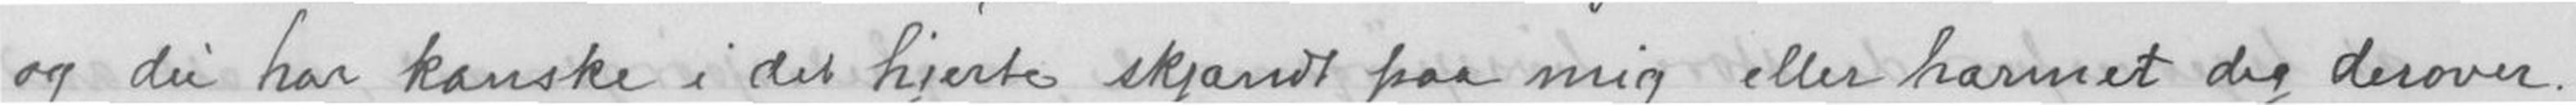og du har kanske i dit hjerte skjændt paa mig eller harmet dig derover.
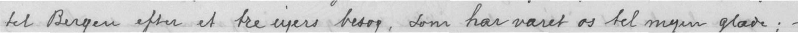til Bergen efter et tre ugers besøg, som har været os til megen glæde; -
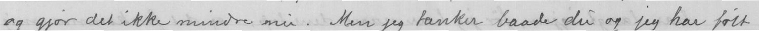og gjør det ikke mindre nu. Men jeg tænker baade du og jeg har følt
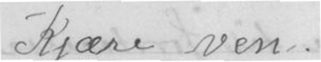Kjære ven.
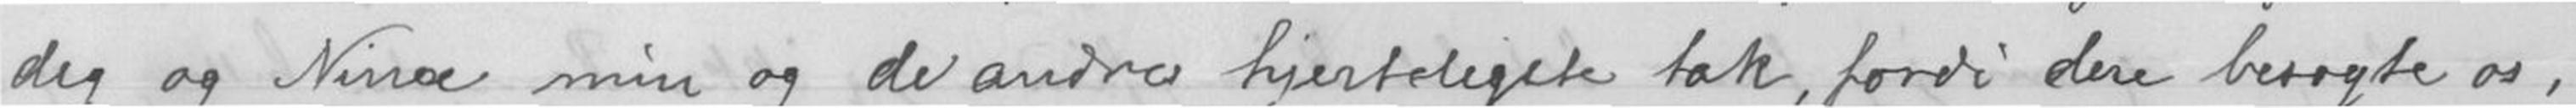dig og Nina min og de andre hjertelegste tak, fordi dere besøgte os,
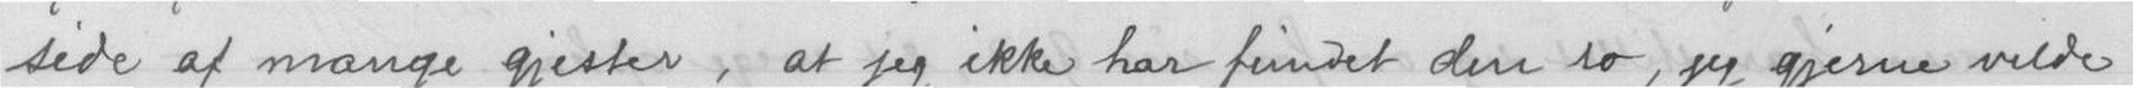side af mange gjester, at jeg ikke har fundet den ro, jeg gjerne vilde
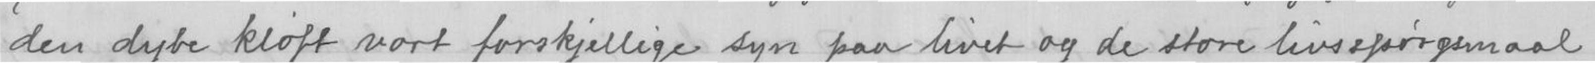den dybe kløft vort forskjellige syn paa livet og de store livsspørgsmaal
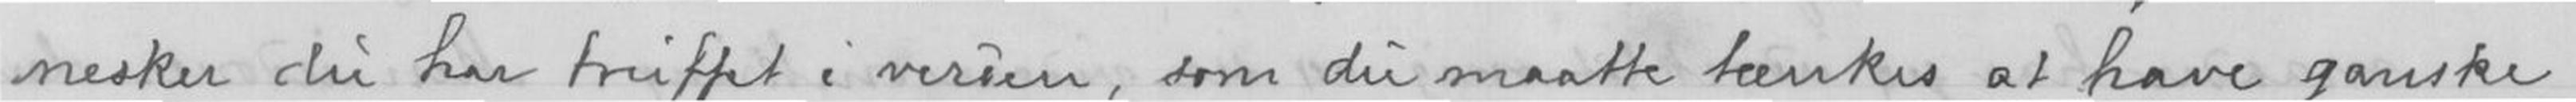nesker du har truffet i verden, som du maatte tænkes at have ganske
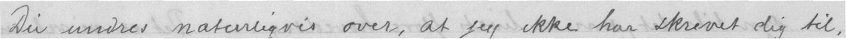Du undres naturligvis over, at jeg ikke har skrevet dig til,
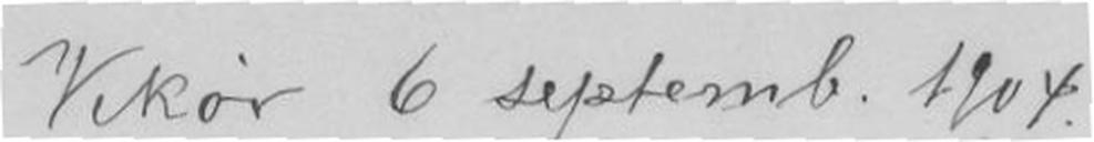Vikør 6 septemb. 1904.
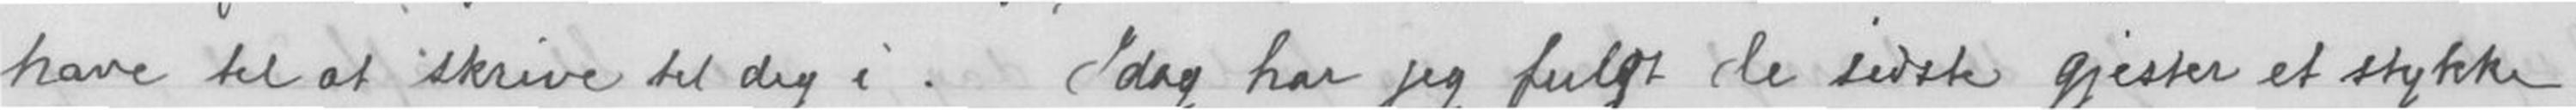have til at skrive til dig i. Idag har jeg fulgt de sidste gjester et stykke
